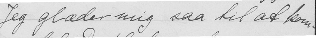Jeg glæder mig saa til at kom-
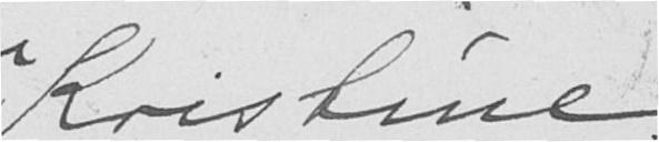Kristine.
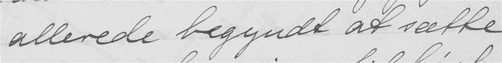allerede begyndt at sætte
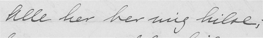Alle her ber mig hilse;
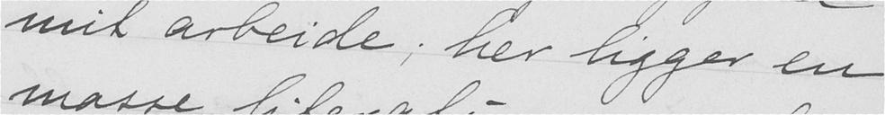mit arbeide; her ligger en
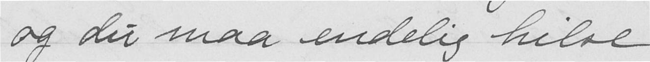og du maa endelig hilse
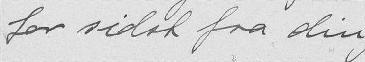for sidst fra din
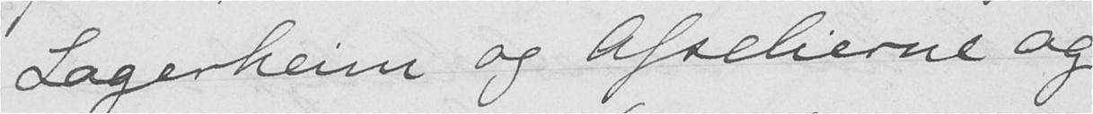Lagerheim og Afselierne og
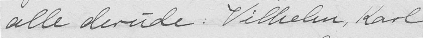alle derude. Vilhelm, Karl
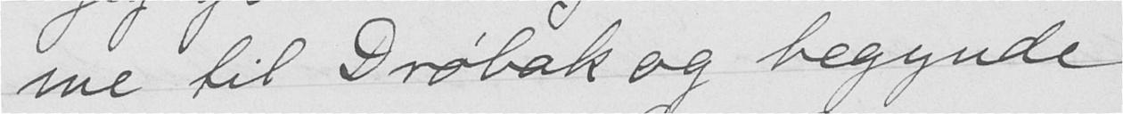me til Drøbak og begynde
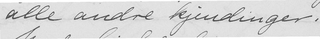alle andre kjendinger.
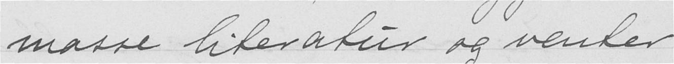masse literatur og venter
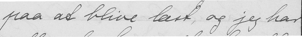paa at blive læst, og jeg har
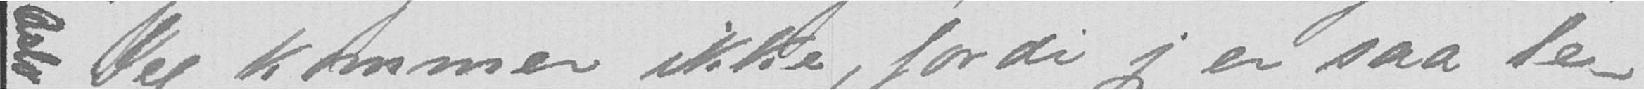Jeg kommer ikke, fordi jeg er saa le-
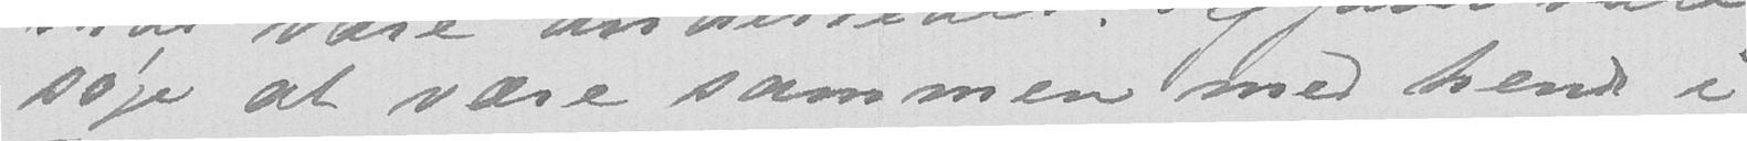søge at være sammen med hende i
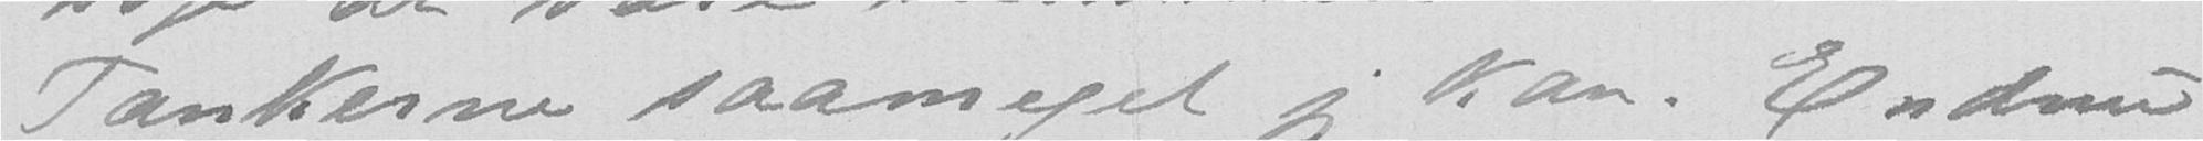Tankerne saameget jeg kan. Endnu
